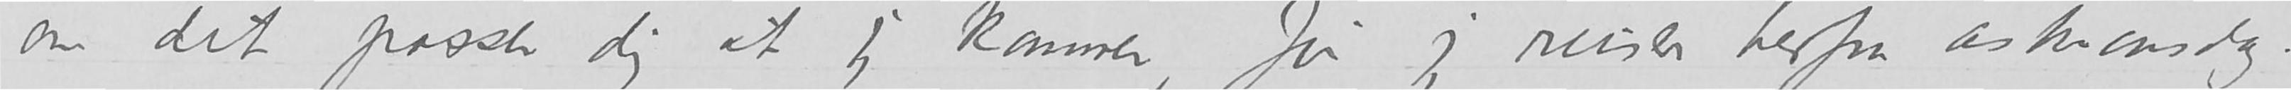om det passer dig at jeg kommer, for jeg reiser herfra askeonsdag.
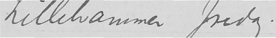Lillehammer fredag.
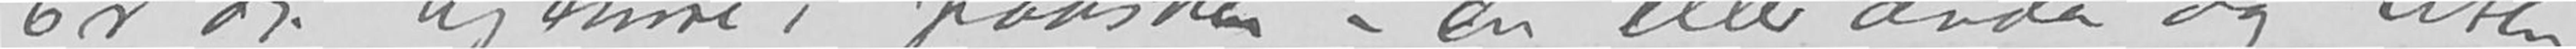Er du hjemme i paasken – en eller anden dag uten
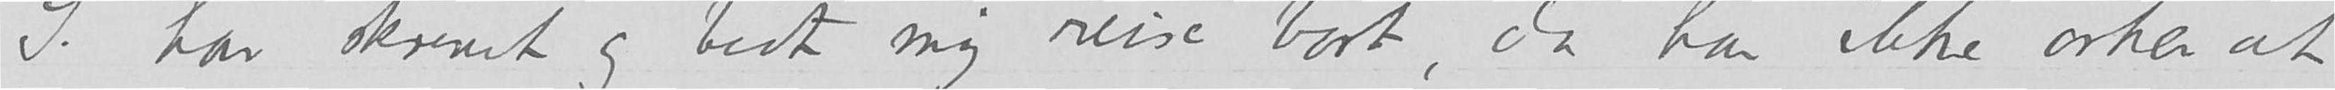S. har skrevet og bedt mig reise bort, da han ikke orker at
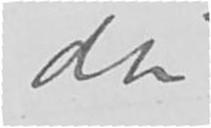din
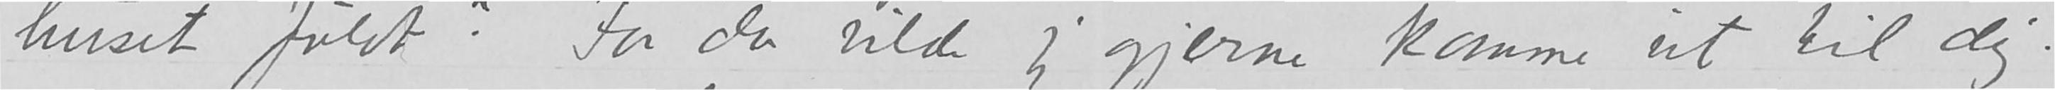huset fuldt?  For da vilde jeg gjerne komme ut til dig.
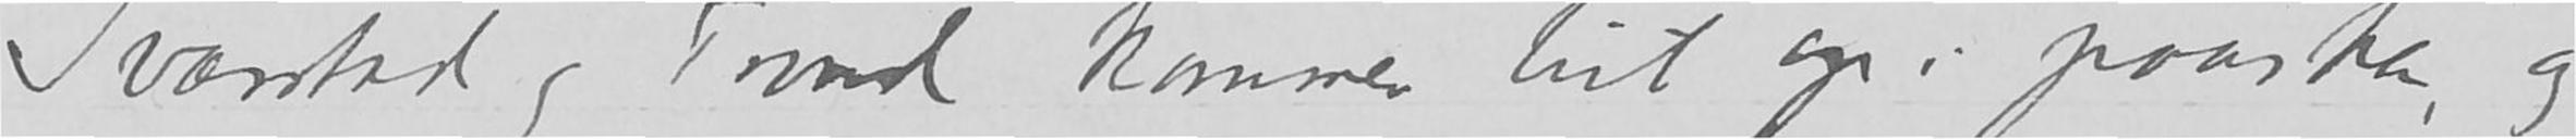Svarstad og Trond kommer hit op i paasken, og
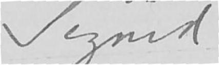Sigrid
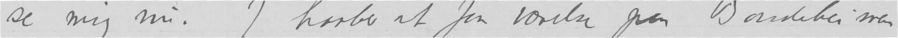se mig nu.  Jeg haaber at faa værelse paa Bondeheimen
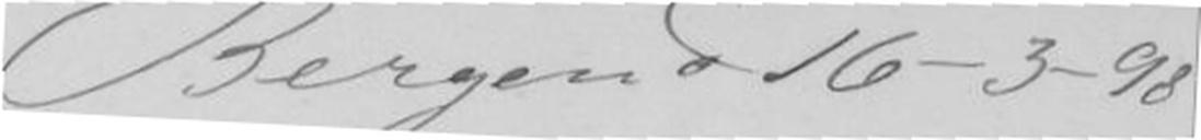Bergen d. 16-3-98
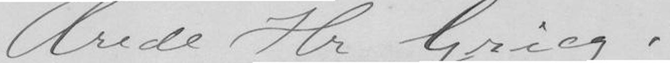Ærede Hr Grieg!
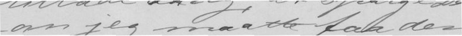- om jeg maatte faa den
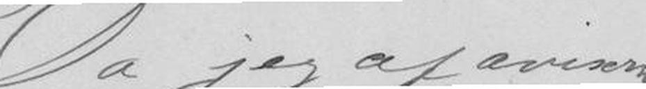Da jeg af aviserne
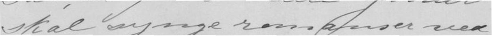skal synge romanser ved
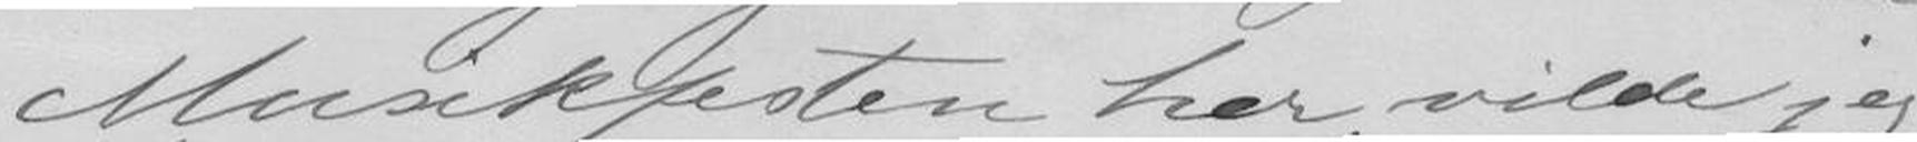Musikfesten her, vilde jeg
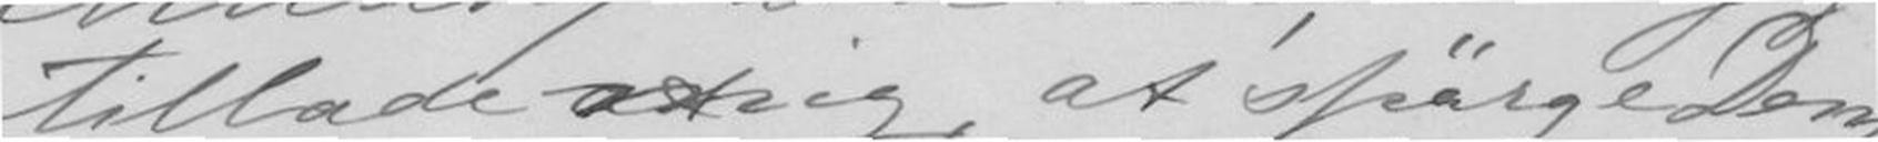tillade mig at spørge Dem
